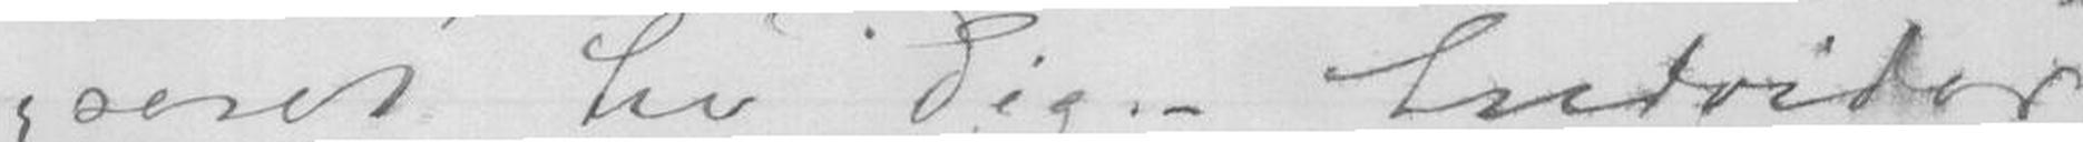seret til Dig. - Endvider
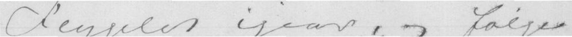Flygelet igaar, og følge
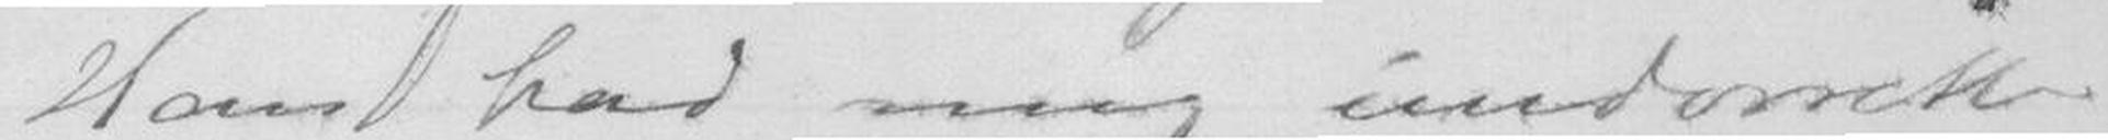Han bad mig underrette
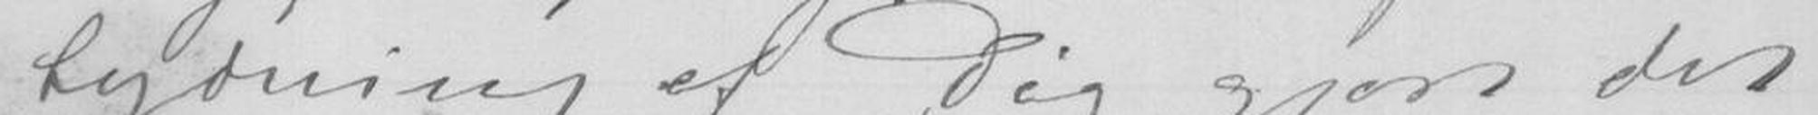tydning af Dig gjort det
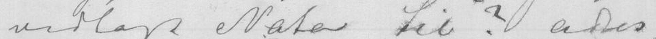vedlagt Noter til? adres-
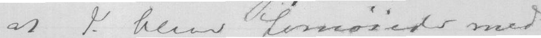at I. bliver fornøiede med
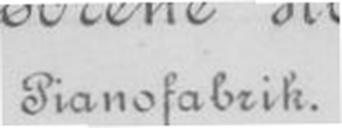Pianofabrik.
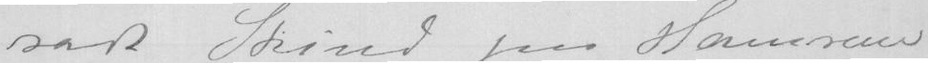sadt Skind paa Hamrene
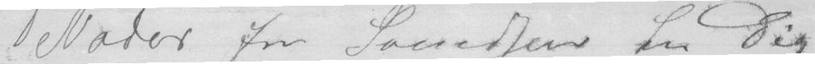Noder fra Svendsen til Dig
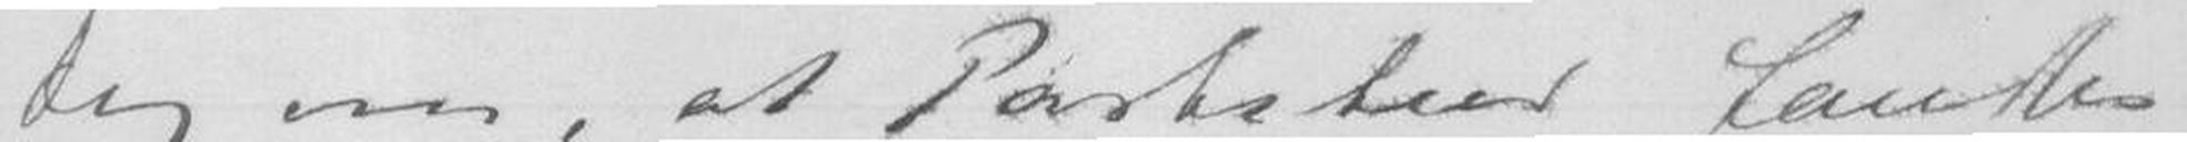Dig om, at Partituret fanttes
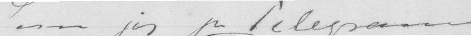Som jeg pr Telegram
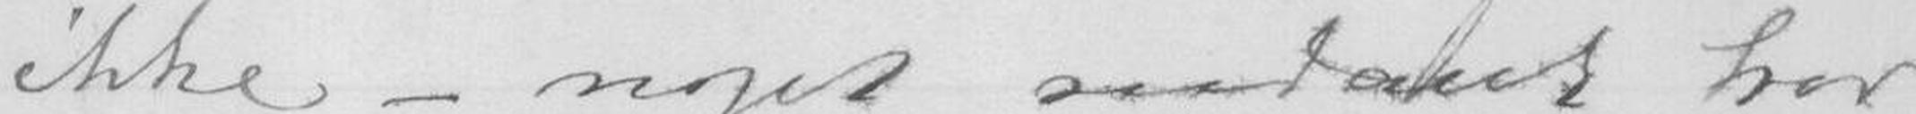ikke - noget saadant tror
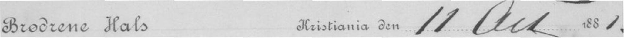Brødrene Hals Kristiania den 11 Oct 1881.
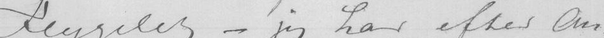Flygelet - jeg har efter An-
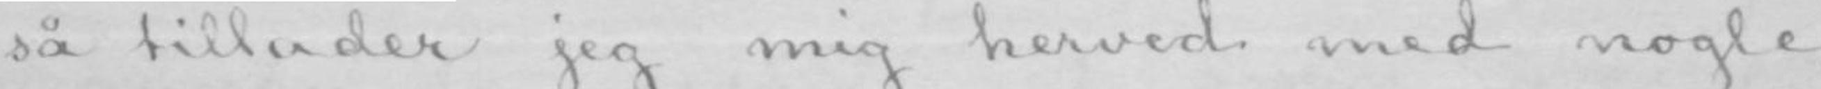så tillader jeg mig herved med nogle
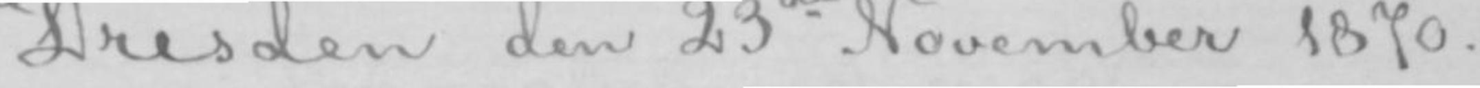Dresden den 23de November 1870.-
